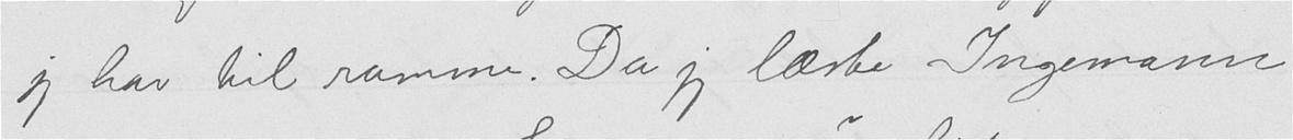jeg har til ramme. Da jeg læste Ingemann
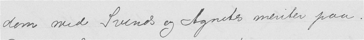dom med Svend og Agnetes meriter paa.
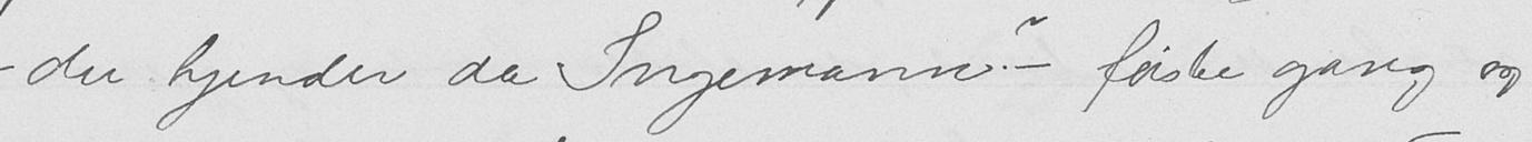- du kjender da Ingemann? - første gang og
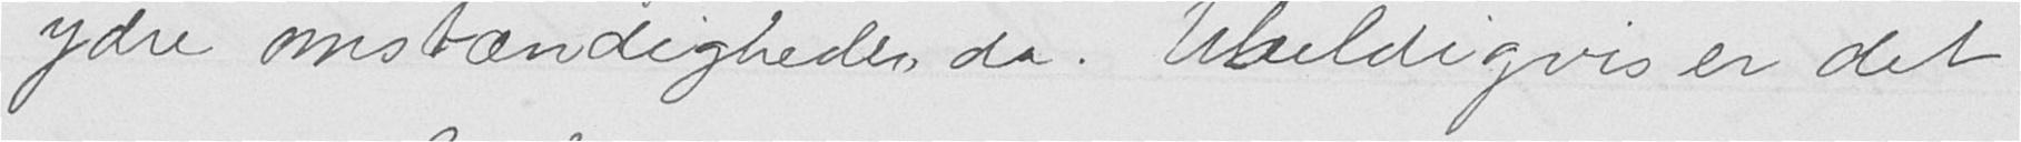ydre omstændigheder da. Uheldigvis er det
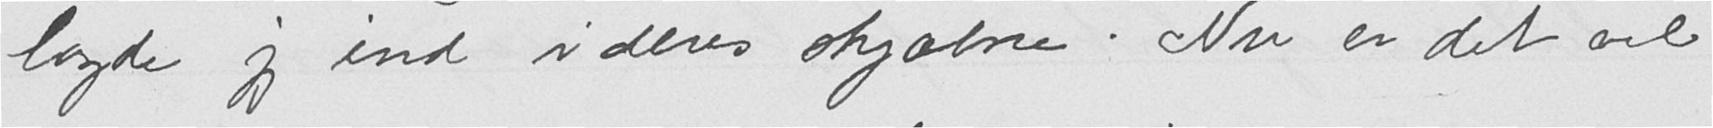lagde jeg ind i deres skjæbne. Nu er det vel
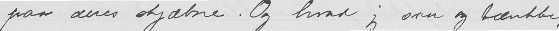paa deres skjæbne. Og hvad jeg saa og tænkte,
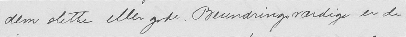dem slette eller gode. Beundringsværdige er de
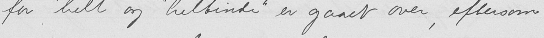for "helt" og "heltinde" er gaaet over, eftersom
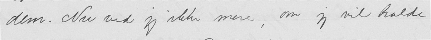dem. Nu ved jeg ikke mere, om jeg vil holde
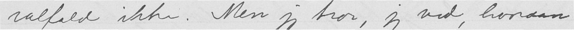ialfald ikke. Men jeg tror, jeg ved, hvordan
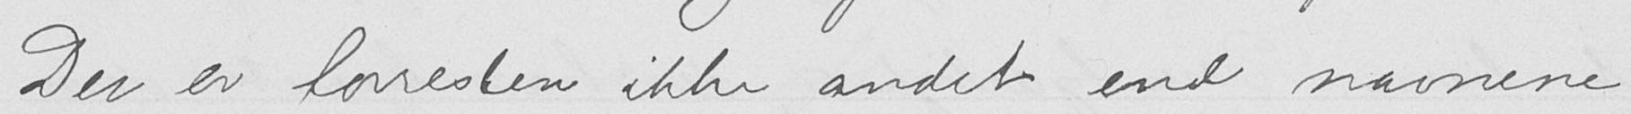Der er forresten ikke andet end navnene
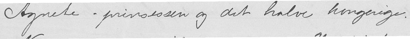Agnete - prinsessen og det halve kongerige.
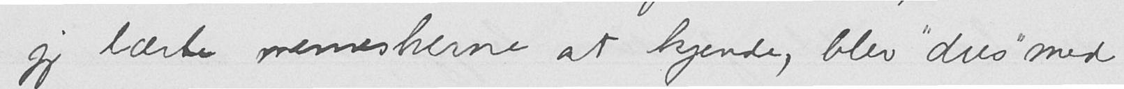jeg lærte menneskerne at kjende, blev "dus" med
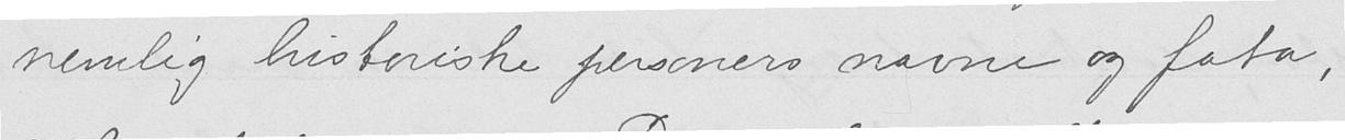nemlig historiske personers navne og fata,
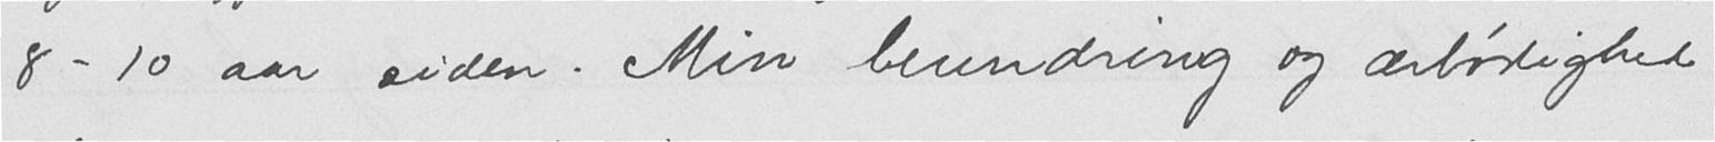8 - 10 aar siden. Min beundring og ærbødighed
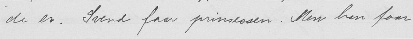de er. Svend faar prinsessen. Men han faar
